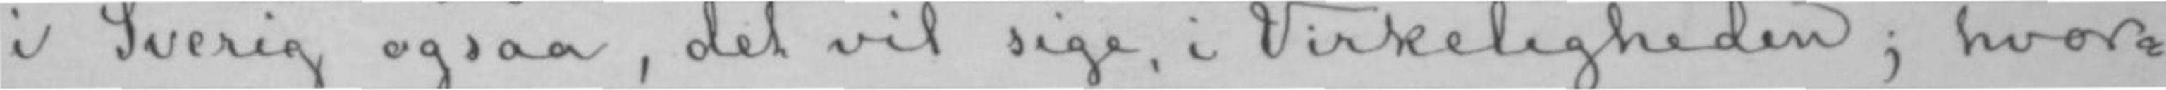i Sverig ogsaa, det vil sige, i Virkeligheden; hvor-
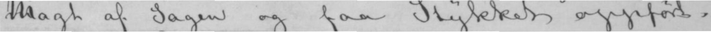Magtmagt af Sagen og faa Stykket oppført.
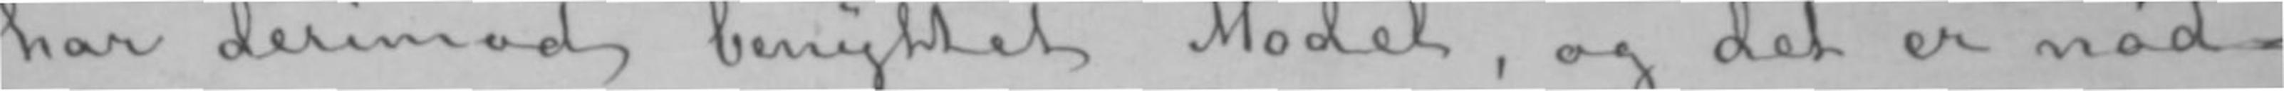har derimod benyttet Model, og det er nød-
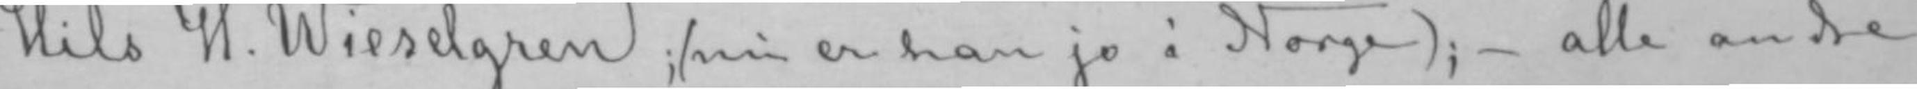Hils H. Wieselgren; (nu er han jo i Norge);- alle andre
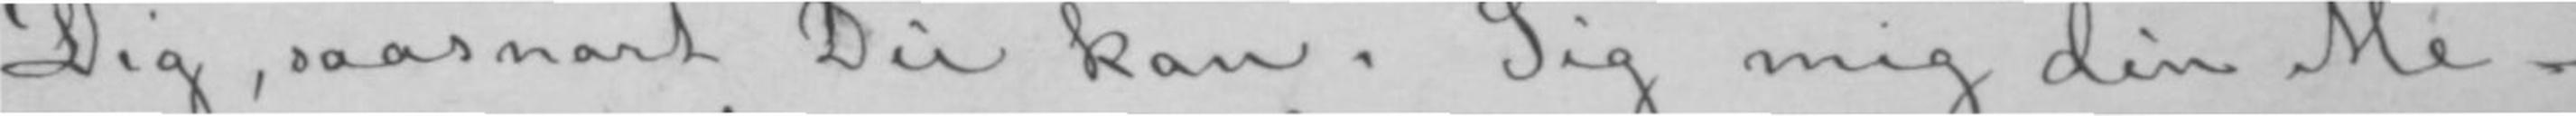Dig, saasnart Du kan. Sig mig din Me-
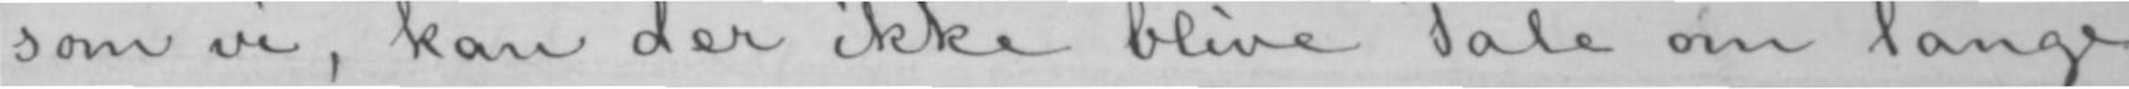som vi, kan der ikke blive Tale om lange
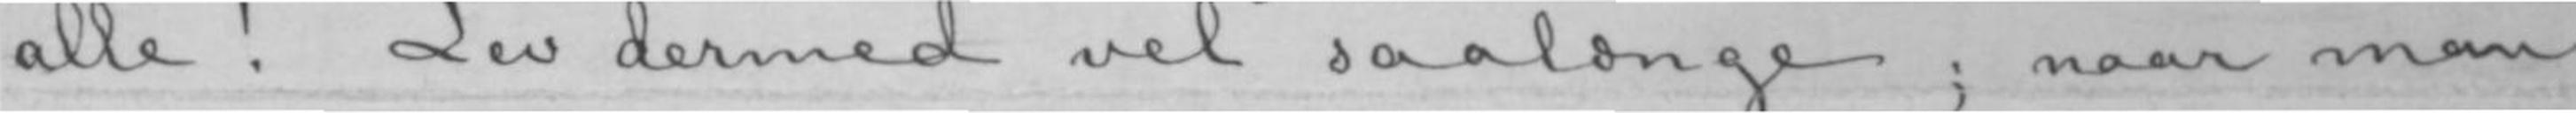alle! Lev dermed vel saalænge; naar man
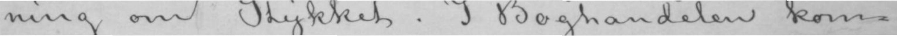ning om Stykket. I Boghandelen kom-
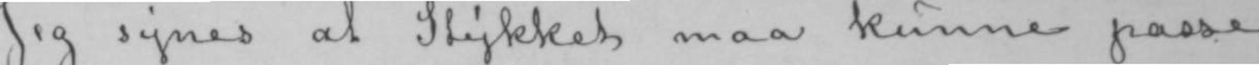Jeg synes at Stykket maa kunne passe
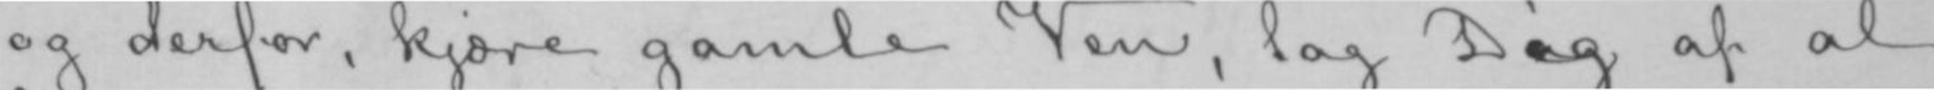og derfor, kjære gamle Ven, tag Dig af al
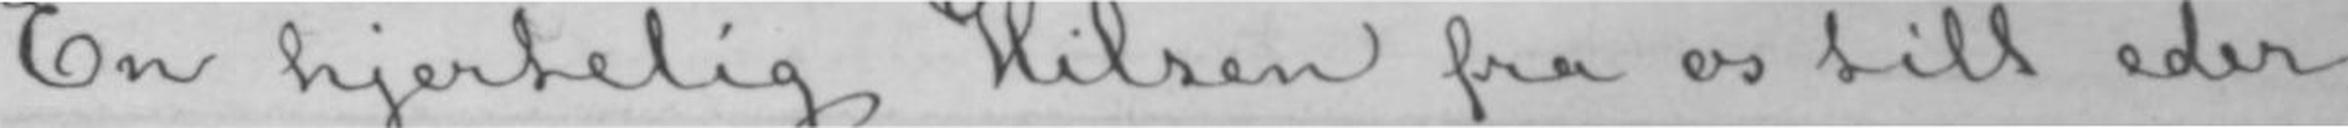En hjertelig Hilsen fra os till eder
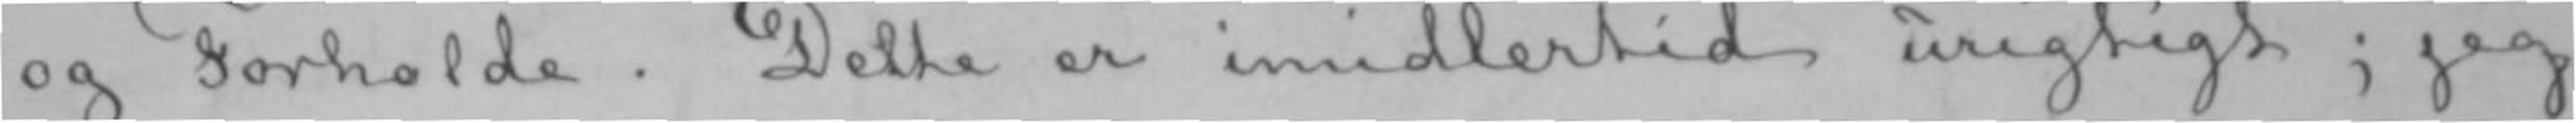og Forholde. Dette er imidlertid urigtigt; jeg
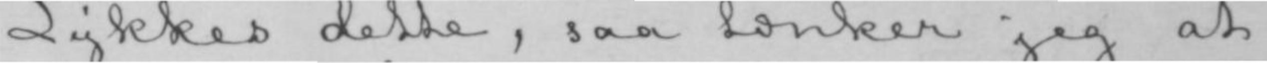Lykkes dette, saa tænker jeg at
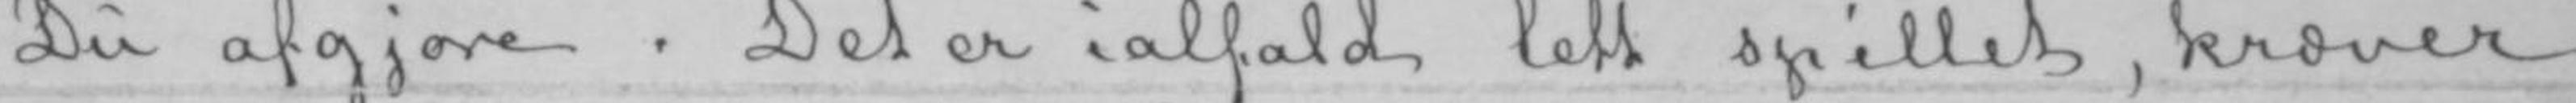Du afgjøre. Det er ialfald lett spillet, kræver
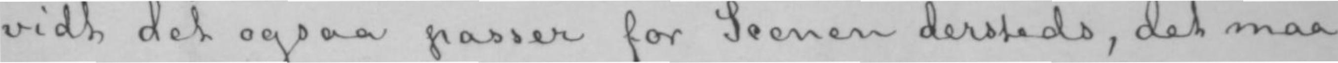vidt det ogsaa passer for Scenen dersteds, det maa
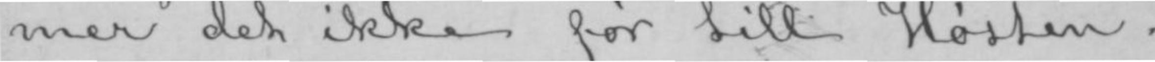mer det ikke før till Høsten.
